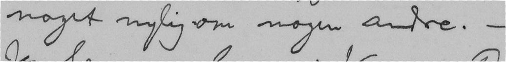noget nylig om nogen andre. -
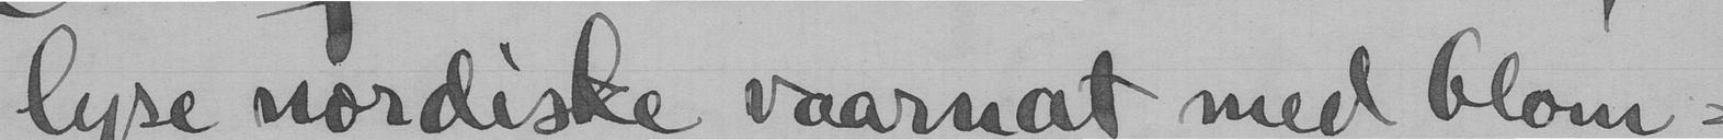lyse nordiske vaarnat med blom-
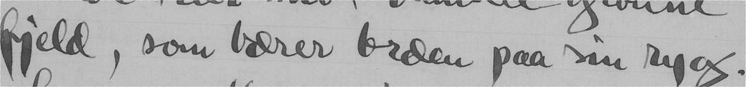fjeld, som bærer bræen paa sin ryg.
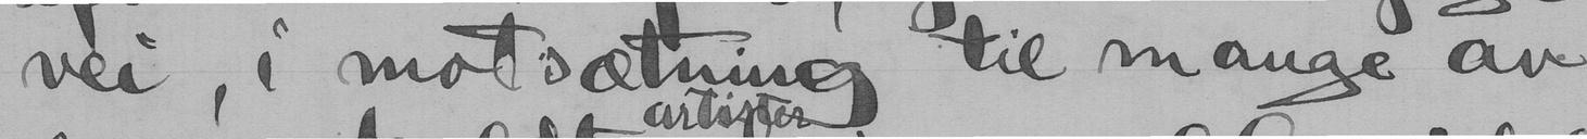vei, i motsætning til mange av
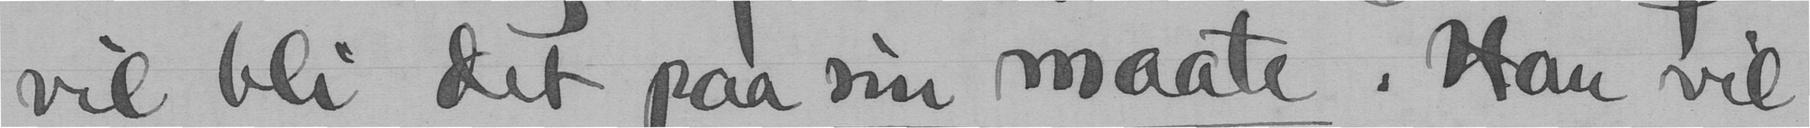vil bli det paa sin maate. Han vil
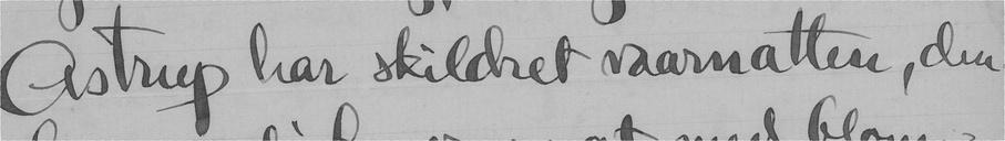Astrup har skildret vaarnatten, den
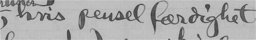, hvis pensel færdighet
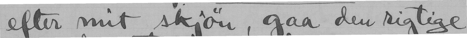efter mit skjøn, gaa den rigtige
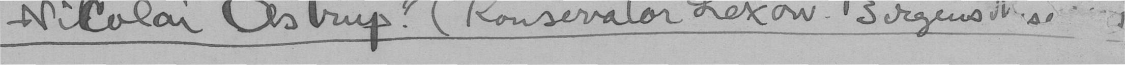Nicolai Astrup". (Konsevator Lexow. Bergens Museum
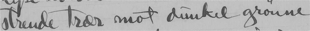strende trær mot dunkel grønne
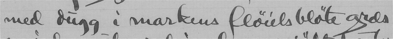med dugg i markens fløielsbløte græs
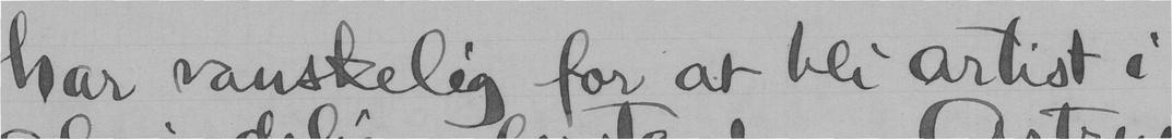har vanskelig for at bli artist i
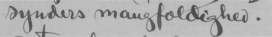synders mangfoldighed.
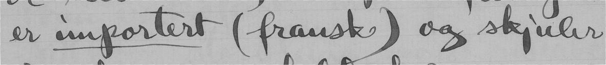er importert (fransk) og skjuler
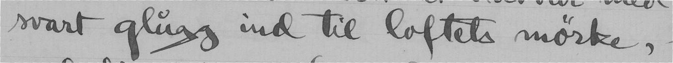svart glugg ind til loftets mørke,
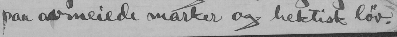paa avmeiede marker og hektisk løv.
